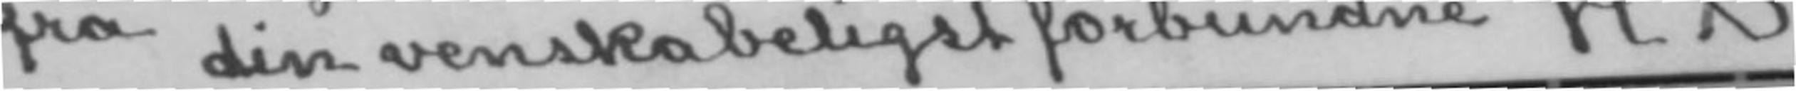fra din venskabeligst forbundne H. I.
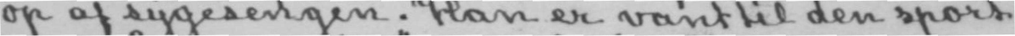op af sygesengen. Han er vant til den sport;
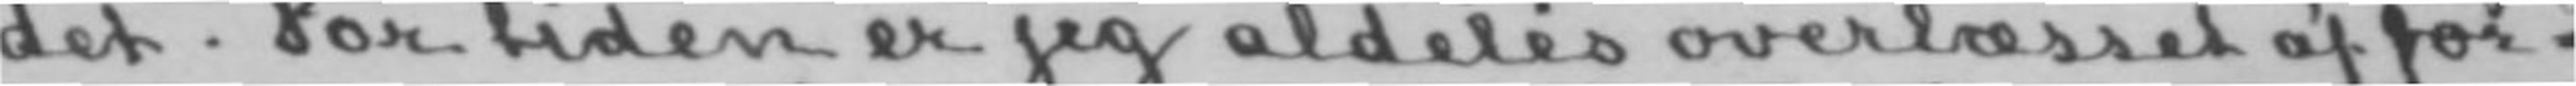det. For tiden er jeg aldeles overlæsset af for-
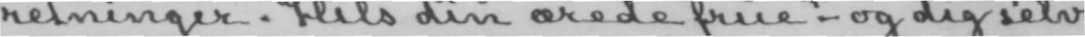retninger. Hils din ærede frue - og dig selv
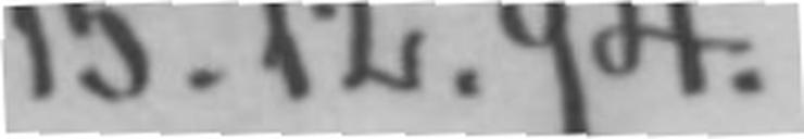15.12.94.
